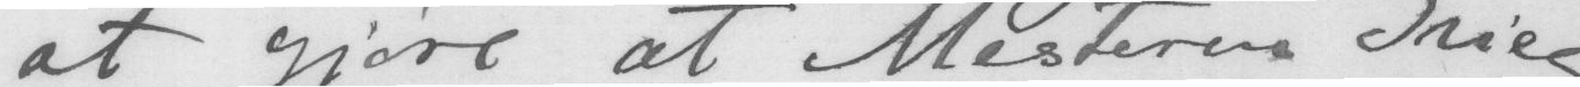at gjøre at mesteren Grieg
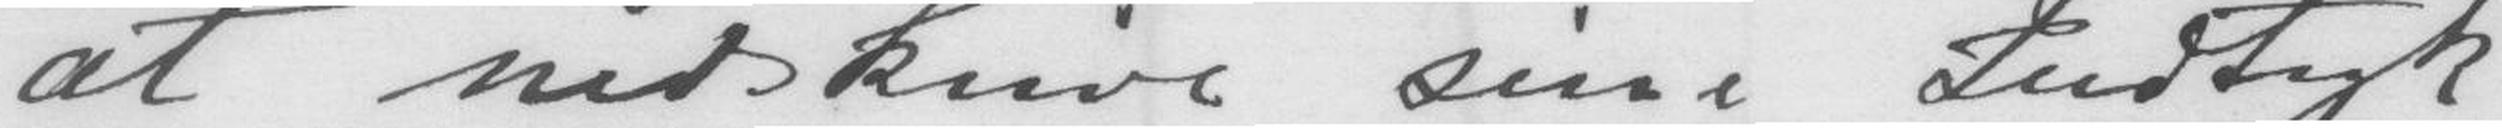at medskrive sine Indtryk
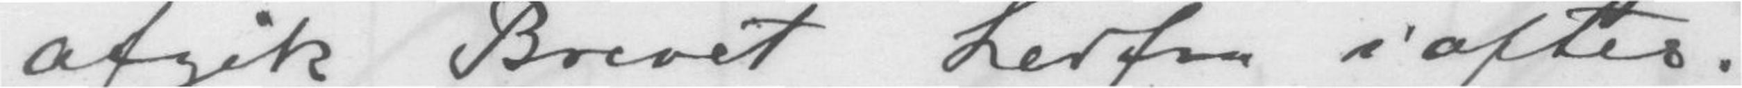afgik Brevet herfra iaftes.
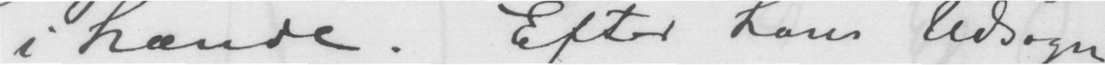i hænde. Efter hans ledsagn
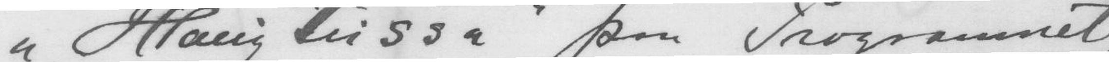"Haugtussa" paa programmet,
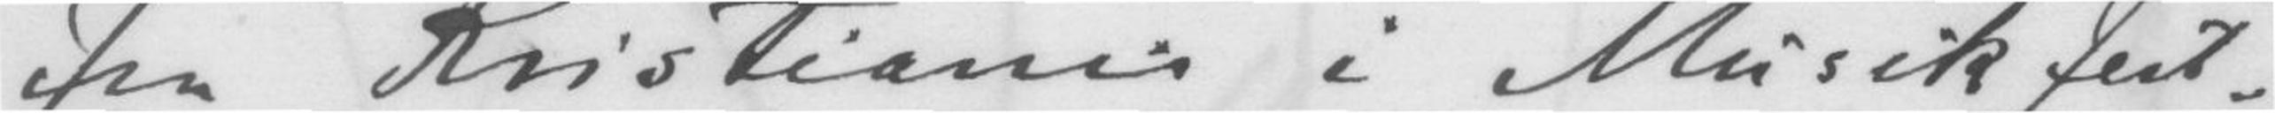fra Kristianias i Musikfest-
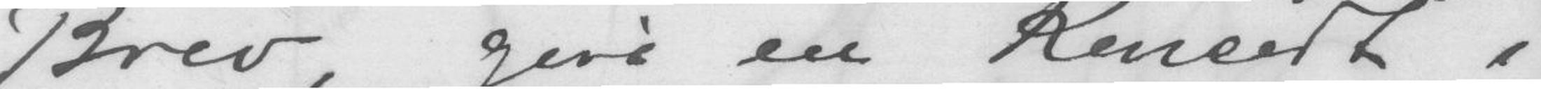Brev, gøre en Koncert i
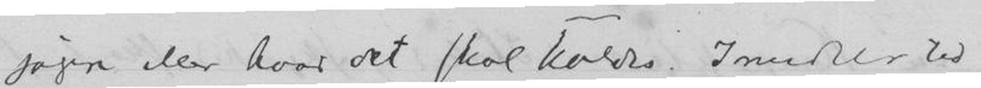sagen eller hvad det skal kaldes. Imidlertid
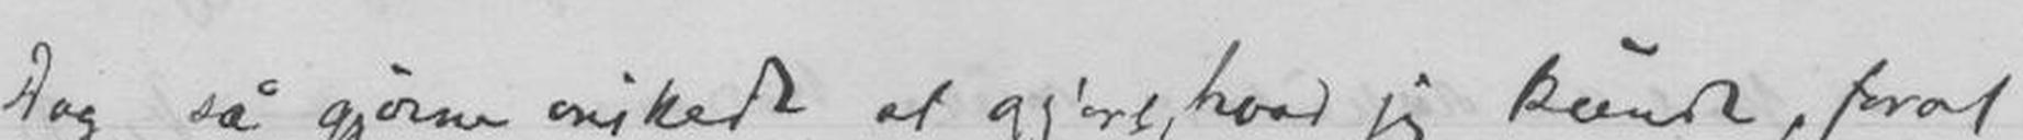Dag så gjerne ønsket at gjøre, hvad jeg kunde, forat
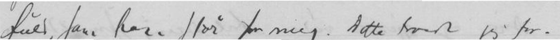fuld som her står for mig. Dette troede jeg for-
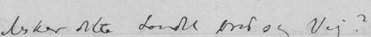elsker dette Landet ond og Vej?
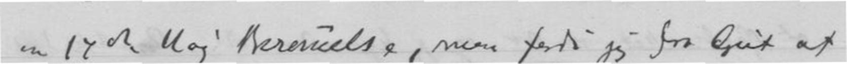en 17de Maj Beruselse, men fordi jeg fra Gut af
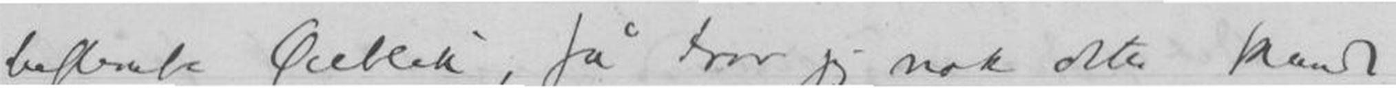bestemte Øieblik, så tror jeg nok dette kunde
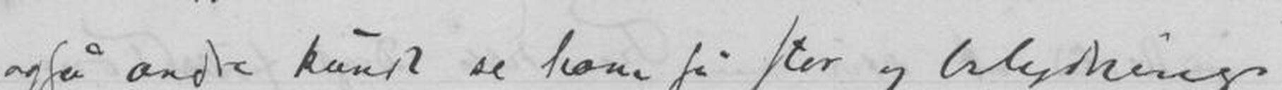også andre kunde se ham få stor og betydnings
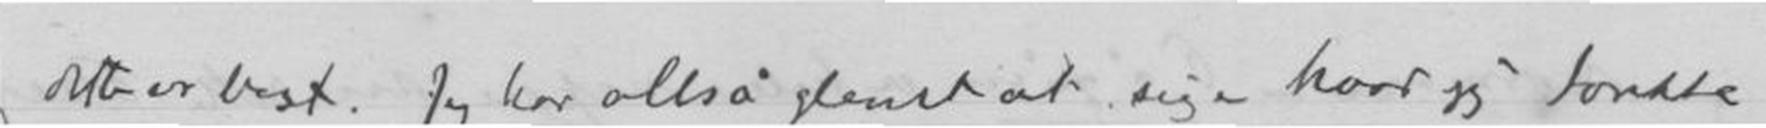dette er bedst. Jeg har altså glemt at sige hvad jeg tænkte
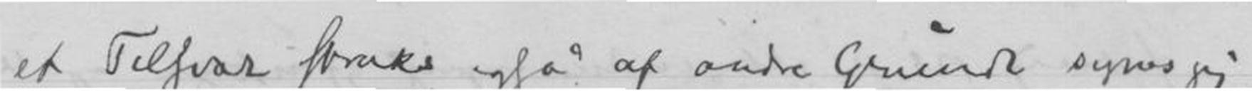et Tilsvar straks også af andre Grunde synes jeg
